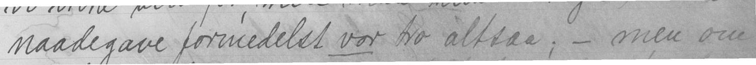naadegave formedelst vor tro altsaa; - men om
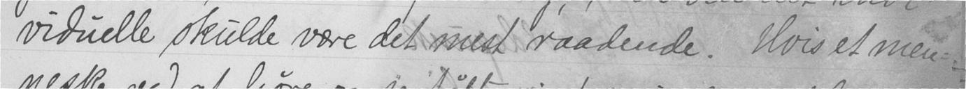viduelle skulde være det mest raadende. Hvis et men-
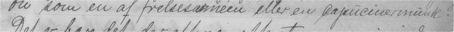on som en af frelsesarméen eller en capucinermunk?
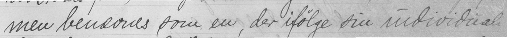men benævnes som en, der ifølge sin individual
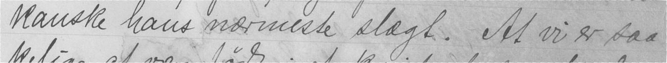kanske hans nærmeste slægt. At vi er saa
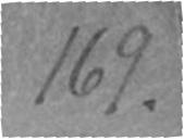169.
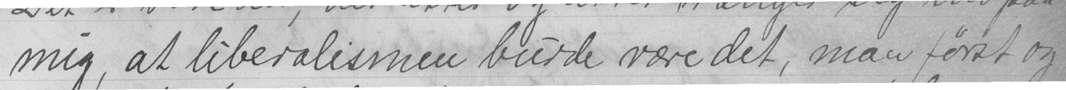mig, at liberalismen burde være det, man først og
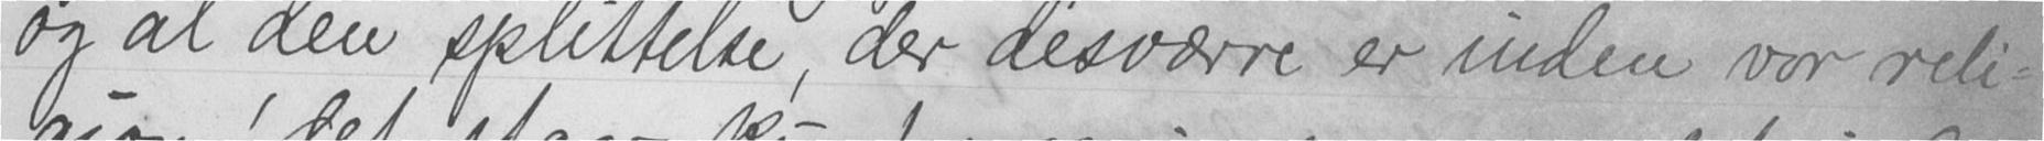og al den splittelse, der desværre er inden vor reli-
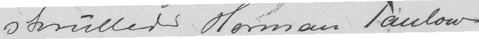skrullede Herman Taulow
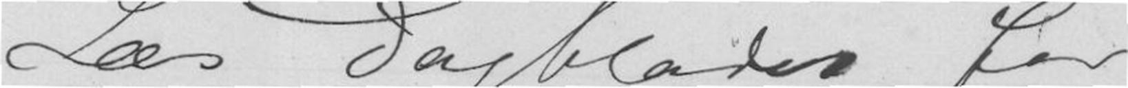Læs Dagbladet for
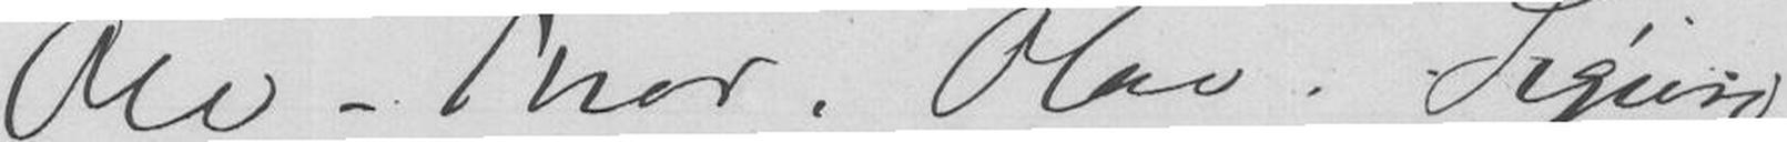Ole, Thor, Olav. Sigurd
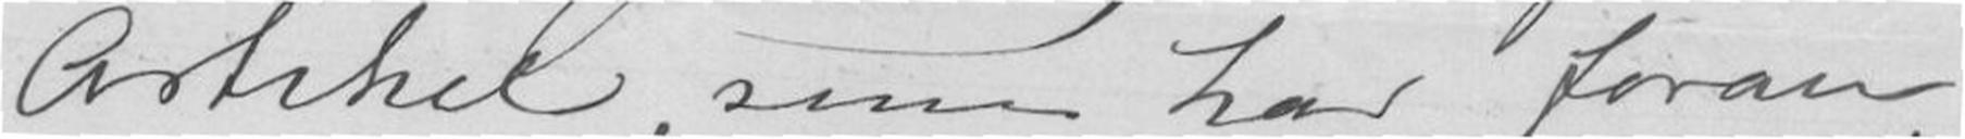Artikel, som har foran
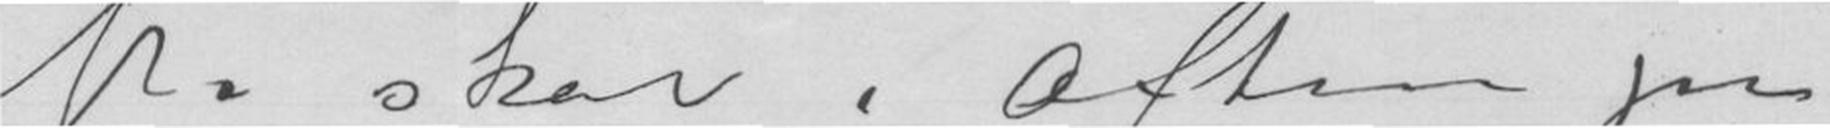Vi skal i Aften paa
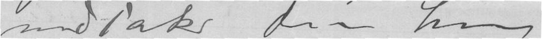med Tak Din heng
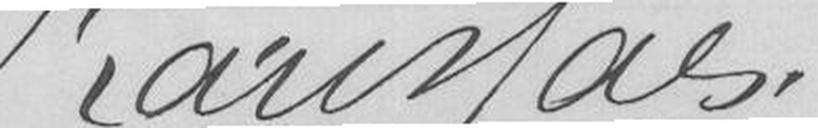Karl Hals
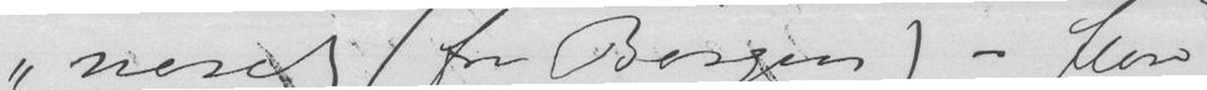deret (fra Bergen) - flere
